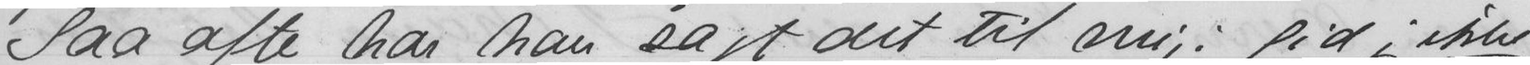Saa ofte har han sagt det til mig. Gid jeg ikke
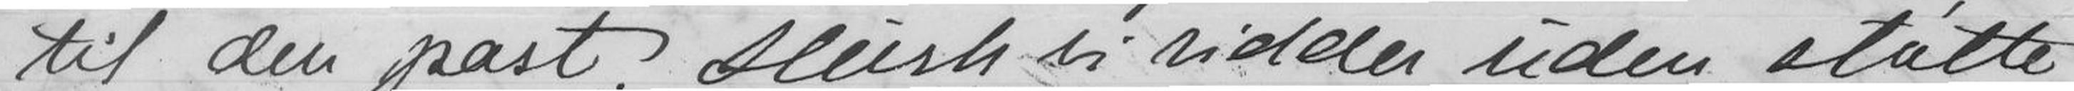til den post. Husk vi sidder uden støtte
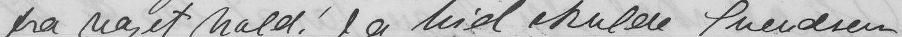fra noget hald! Ja hid skulde Svendsen
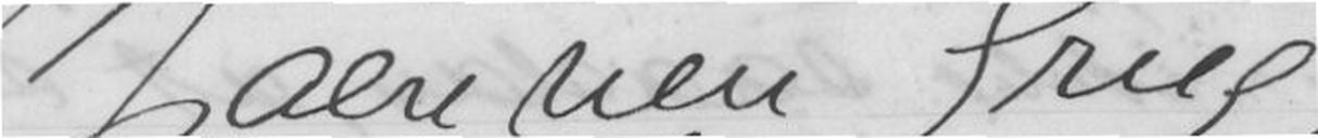Kjære ven Grieg
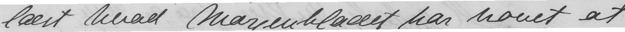læst hvad Morgembladet har havet at
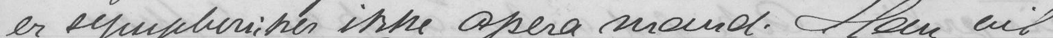er symphoniker ikke opera mand. Han vil
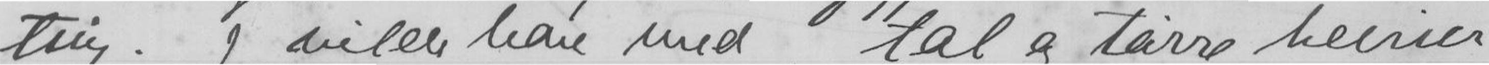ting. Jeg vilde have med tal og tørre beviser
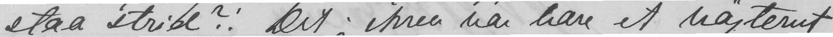staa strid?! Det jeg skrev var bare et nøgternt -
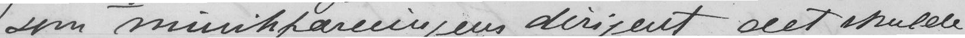som musikforeningens dirigent, det skulde
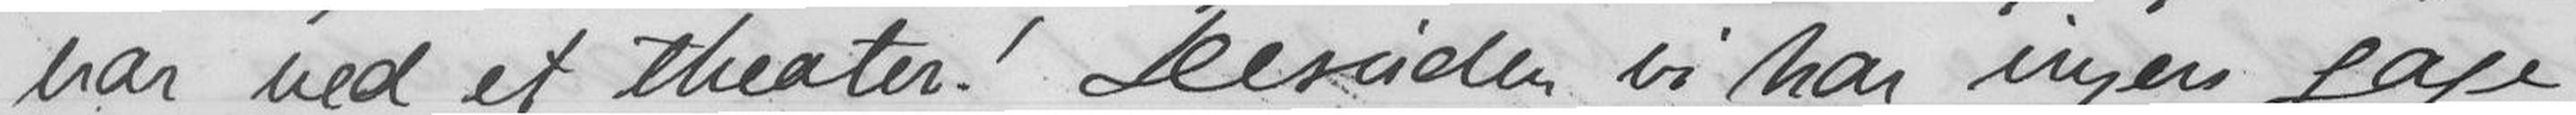var ved et theater! Desuden vi har ingen gage
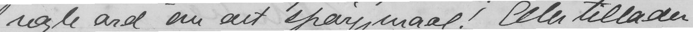nogle ord om det spørgsmaal! Eller tillader
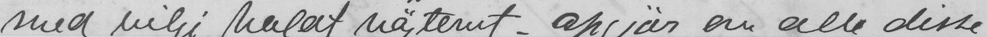med vilje haldt nøgternt - opgjør om alle disse
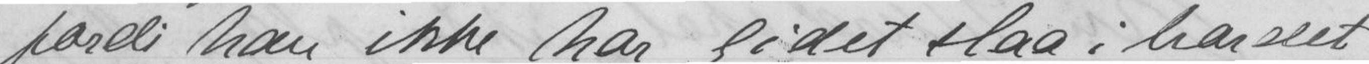fordi ham ikke har gidet slaa i bordet.
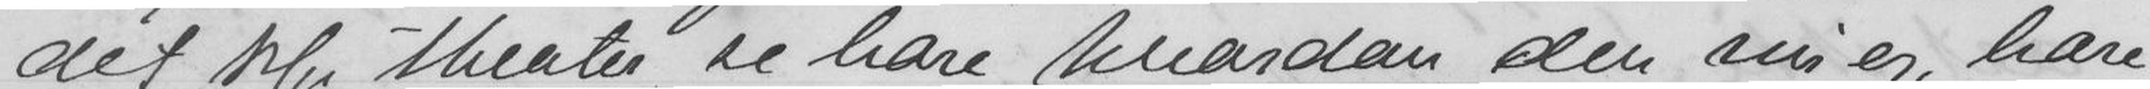det klg theater, til bare hvordan den nu er, bare
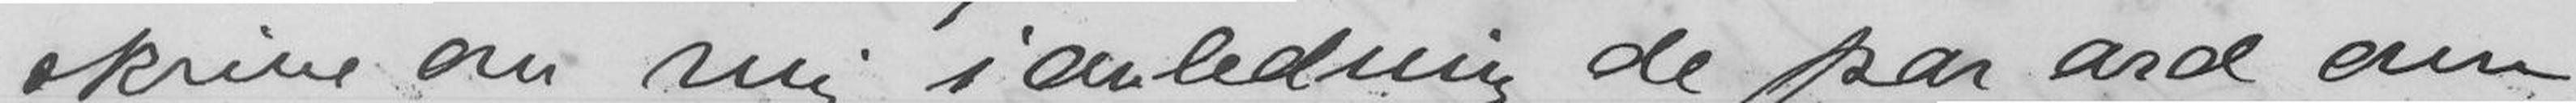skrive om mig i anledning de par ord om
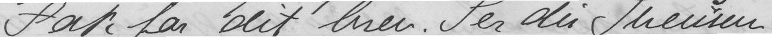Tak for dit brev. Ser du Svendsen
